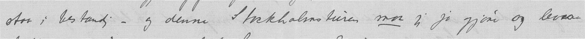staa i bestandig - og denne Stockholmsturen maa jeg jo gjøre og levere
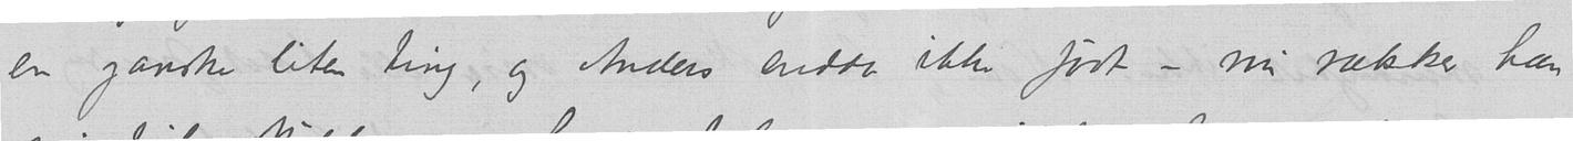en ganske liten ting, og Anders endda ikke født - nå rækker han
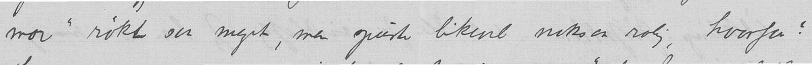mor" røkte saa meget, men spurte likevel noksaa rolig, hvorfor?
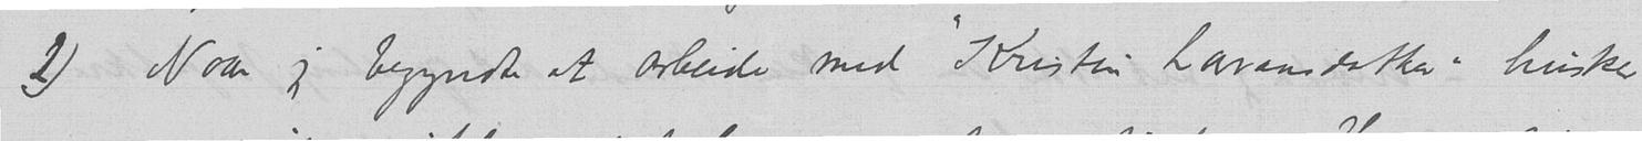2) Naar jeg begyndte at arbeide med "Kristin Lavransdatter" husker
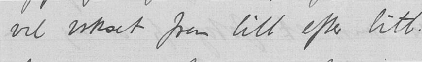vel vokset frem litt efter litt.
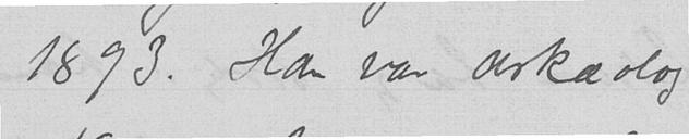1893. Han var arkæolog.
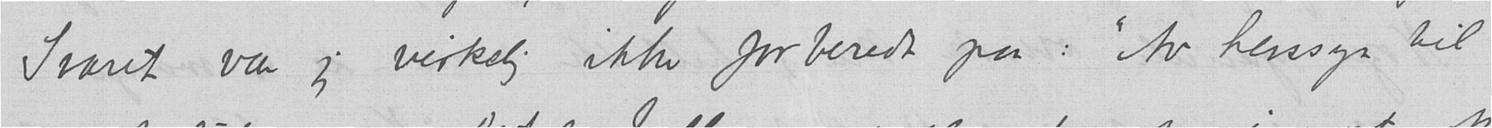Svaret var jeg virkelig ikke forberedt paa: "Av hensyn til
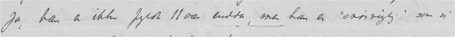Ja, han er ikke fyldt 11 aar endda, men han er "snørvigtig" som vi
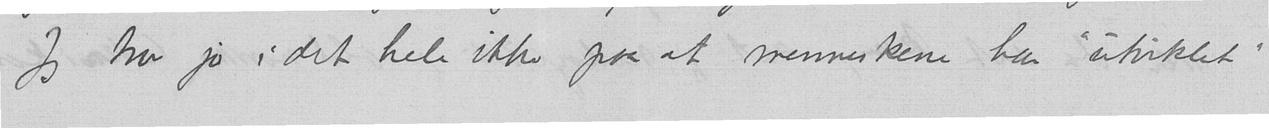Jeg tror jo i det hele ikke paa at menneskene har "utviklet"
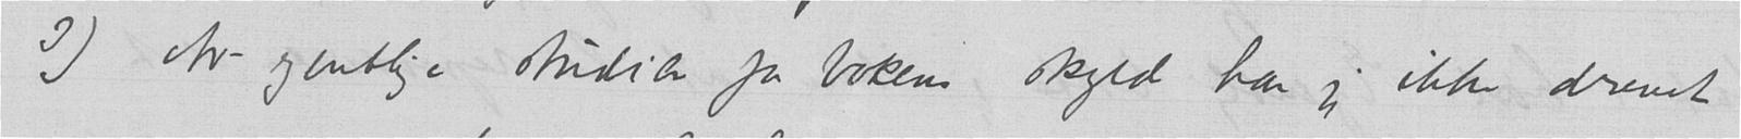3) Av egentlige studier for bokens skyld har jeg ikke drevet
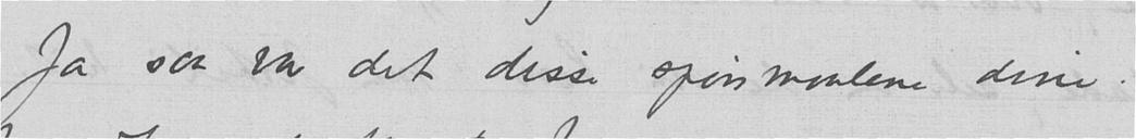Ja saa var det disse spørsmaalene dine.
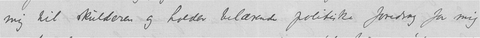mig til skulderen og holder belærende politiske foredrag for mig
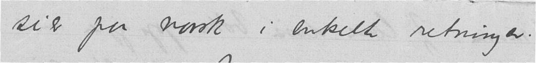sier paa norsk i enkelte retninger.
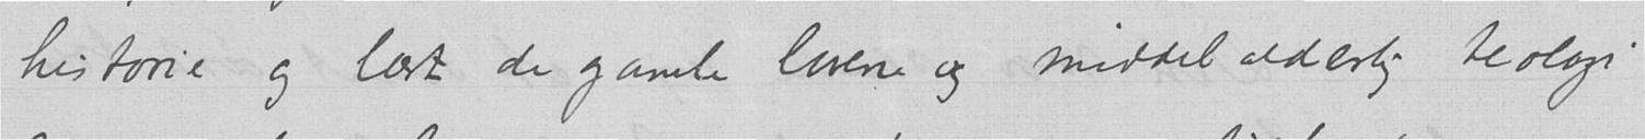historie og læst de gamle lovene og middelalderlig teologi
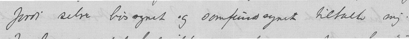fordi selve livssynet og samfundssynet tiltalte mig.
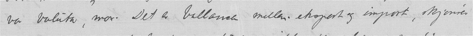vor valuta, mor. Det er ballance mellem eksport og import, skjønner
